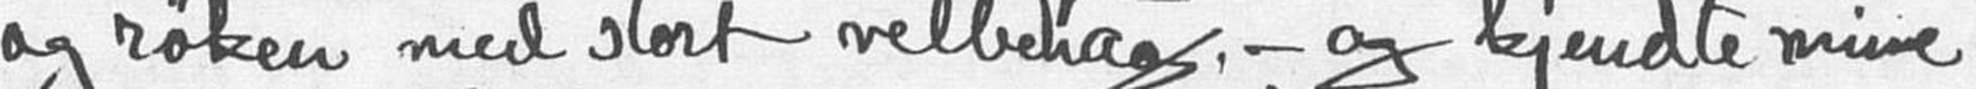og røken med stort velbehag, - og kjendte mine
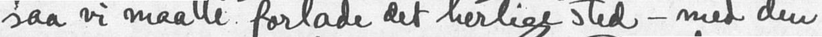saa vi maate forlade det herlige sted - med den
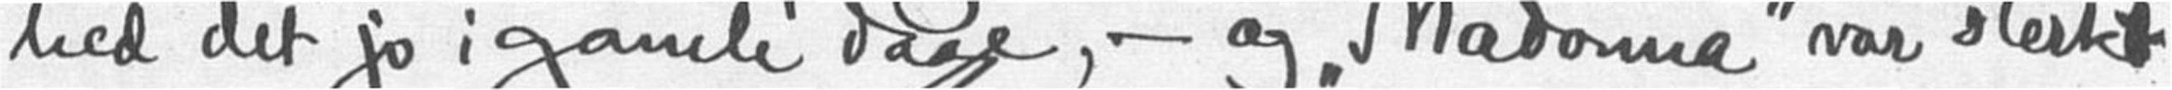hed det jo i gamle dage, - og "Madonna" var strekt
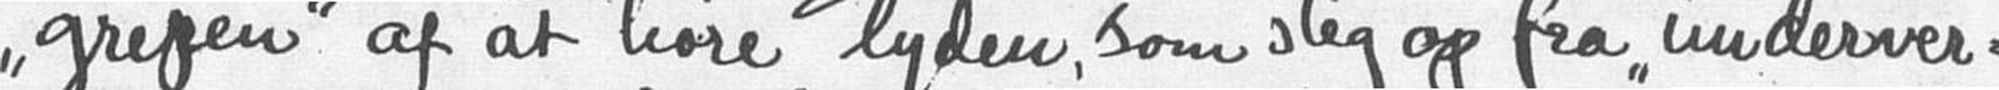"grepen" af at høre lyden, som steg op fra "underver-
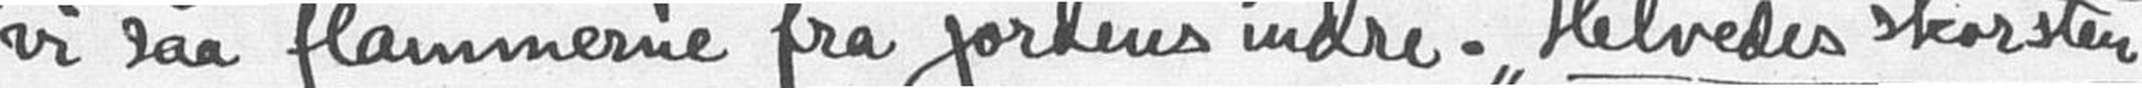vi saa flammerne fra Jordens indre. "Helvedes skorsten"
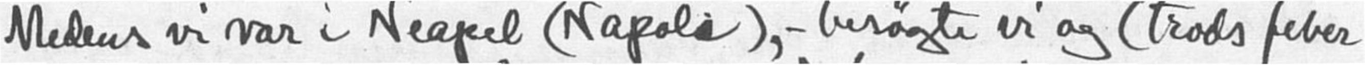Medens vi var i Neapel (Napoli), -besøgte vi og (trods feber
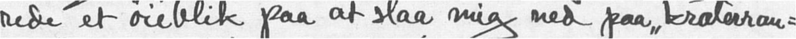rede et øieblik paa at slaa mig ned paa "kraterran-
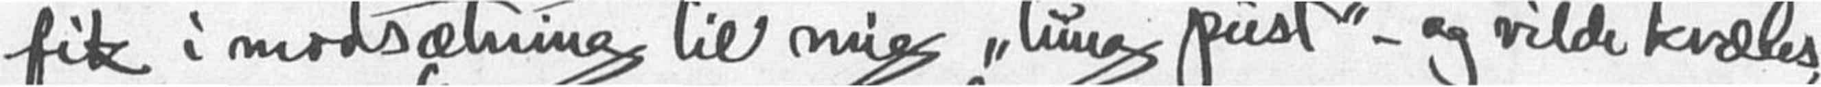fik i modsætning til mig "tung pust" - og vilde kvæles,
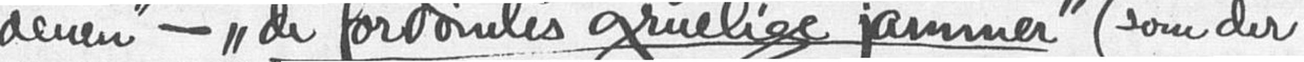denen" - "de fordømtes gruelige jammer" (som der
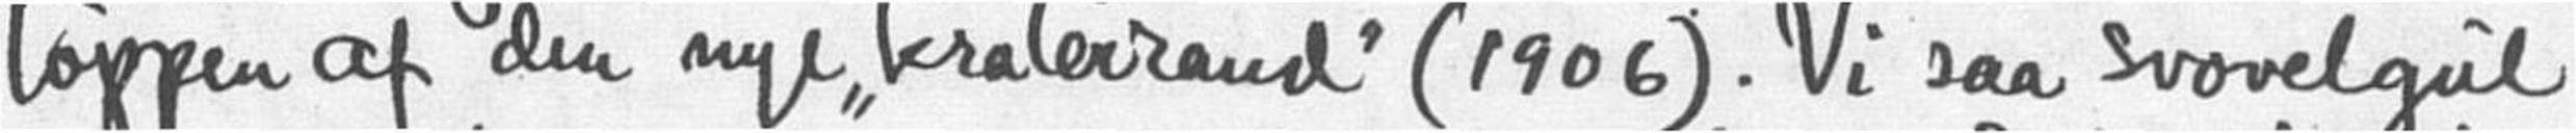toppen af den nye "kraterrand" (1906). Vi saa svovelgul
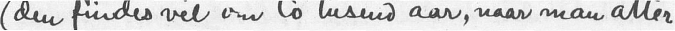(den findes vel om to tusend aar, naar man atter
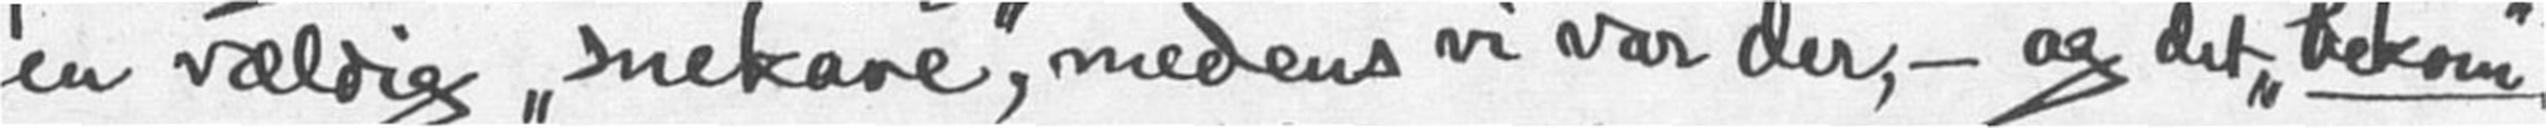en vældig "snekave", medens vi var der, - og det "bekom"
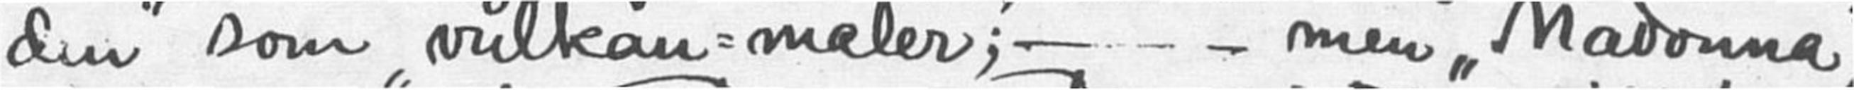den" som "vulkan-maler"; - men "Madonna"
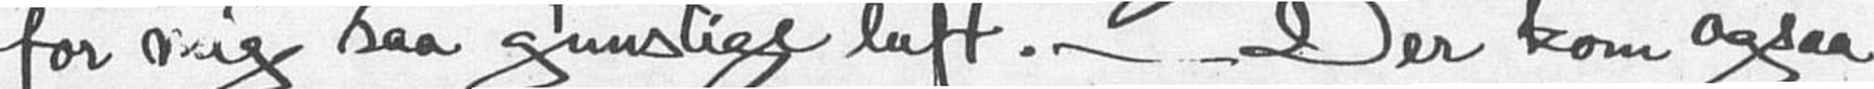for mig saa gunstige luft. - Der kom ogsaa
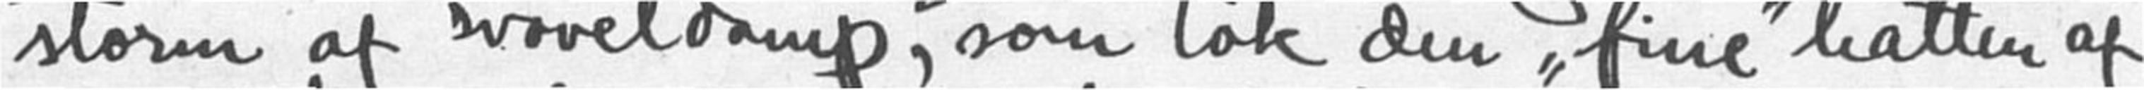storm af svoveldamp, som tok tok den "fine" hatten af
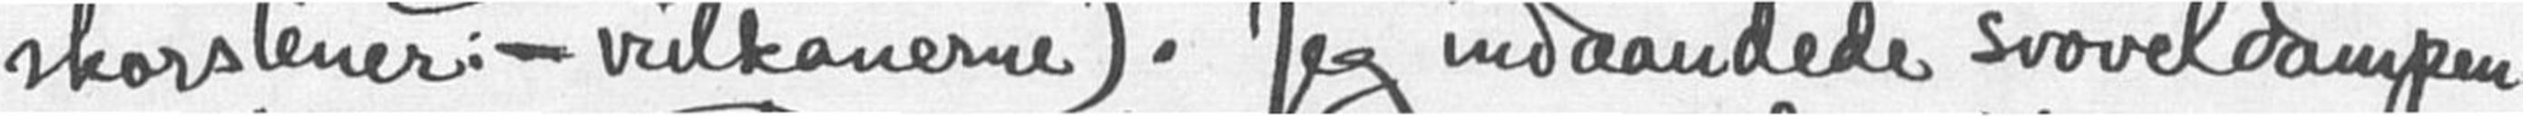skorstener: - vulkanerne). Jeg indaandede svoveldampen
